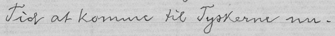Tid at komme til Tyskerne nu.
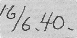16/6. 40.
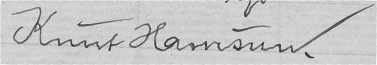Knut Hamsun.
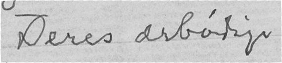Deres ærbødige
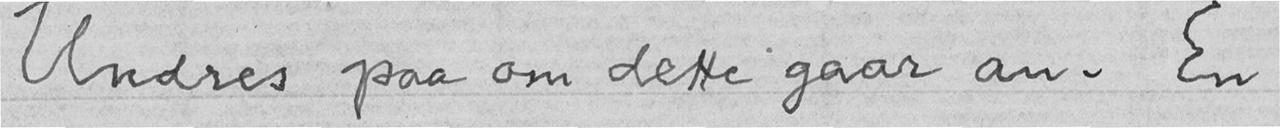Undres paa om dette gaar an. En
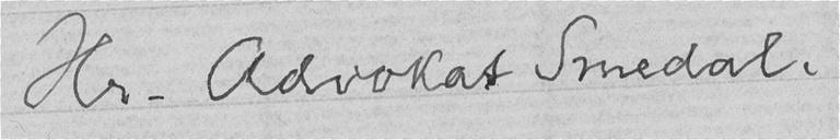Hr. Advokat Smedal.
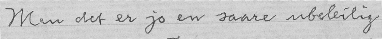Men det er jo en saare ubeleilig
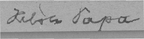Hilsen Papa
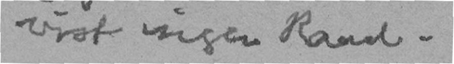vist ingen Raad.
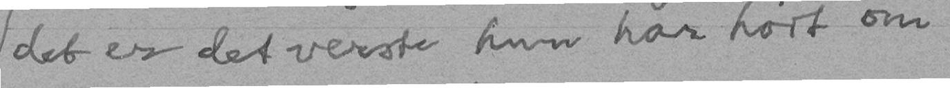det er det verste hun har hørt om
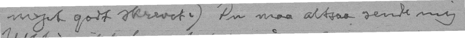meget godt skrevet.) Du maa altsaa sende mig
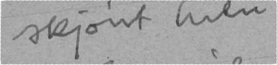skjønt hun
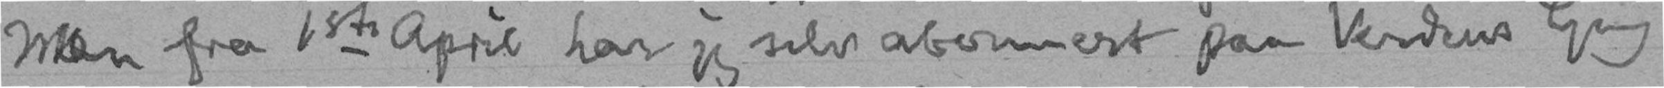Men fra 1ste April har jeg selv abonnert paa Verdens Gang
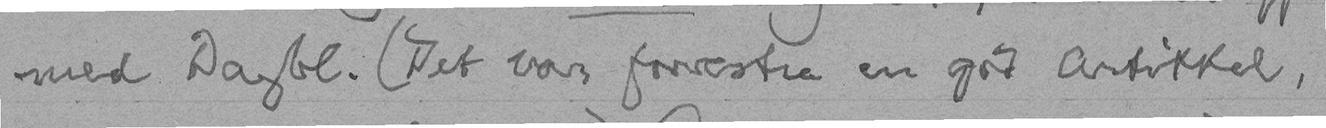med Dagbl. (Det var forresten en god Artikkel,
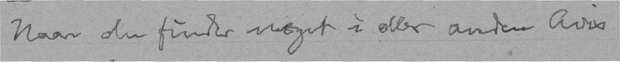Naar du finder noget i eller anden Avis
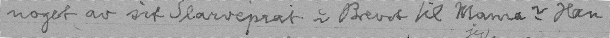noget av sit Slarveprat i Brevet til Mama? Han
